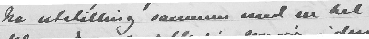ha utstilling sammen med en hel
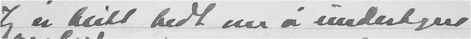Jeg er blitt bedt om å underskrive
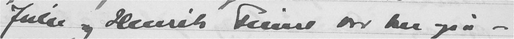Julie og Henrik Finne var her også -
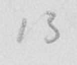13
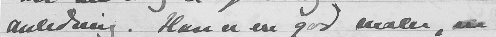anledning. Han er en god maler, en
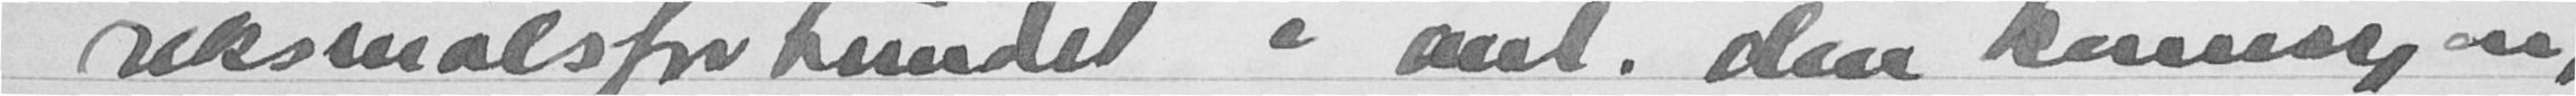riksmålsforbundet i anl. den komisjon,
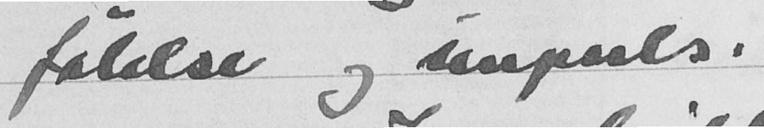følelse og impuls.
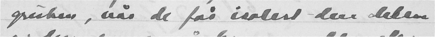gruben, når de får isolert den delen
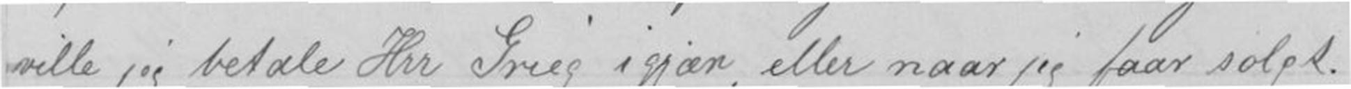ville jeg betale Hrr Grieg igjæn, eller naar jeg faar solgt,
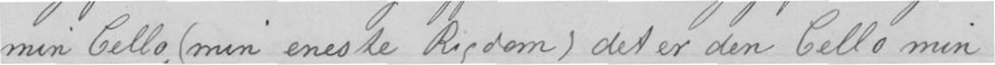min Cello, (min eneste Rigdom) det er den Cello min
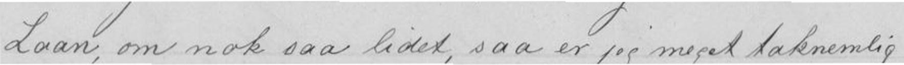Laan, om nok saa lidet, saa er jeg meget taknemlig
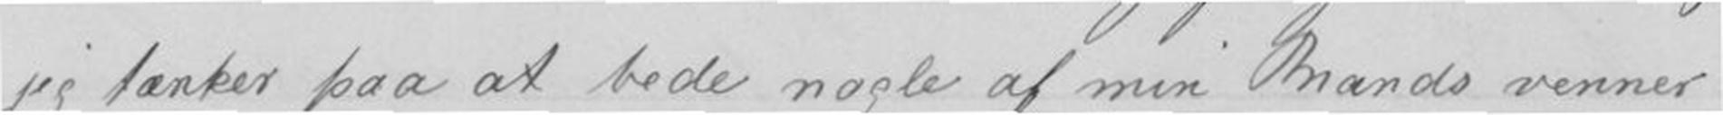jeg tænker paa at bede nogle af min Mands venner
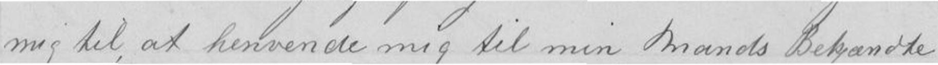mig til at henvende mig til min Mands Bekjændte
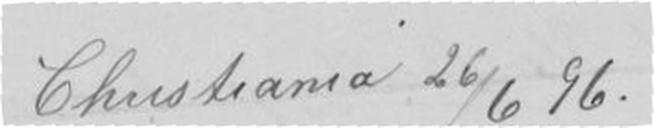Christiania 26/6-96.
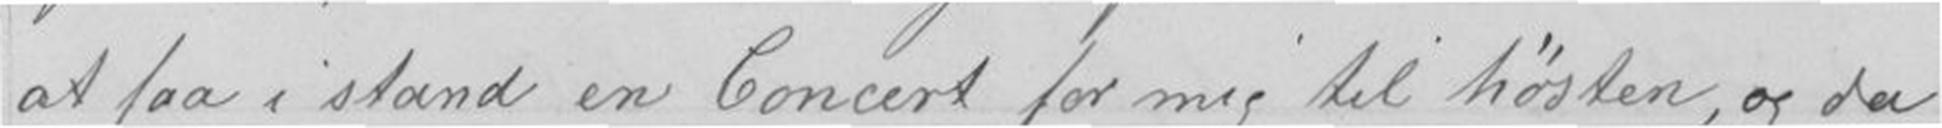at faa i stand en Concert for mig til høsten, og da
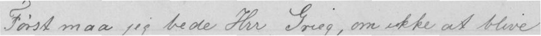Først maa jeg bede Hrr. Grieg, om ikke at blive
